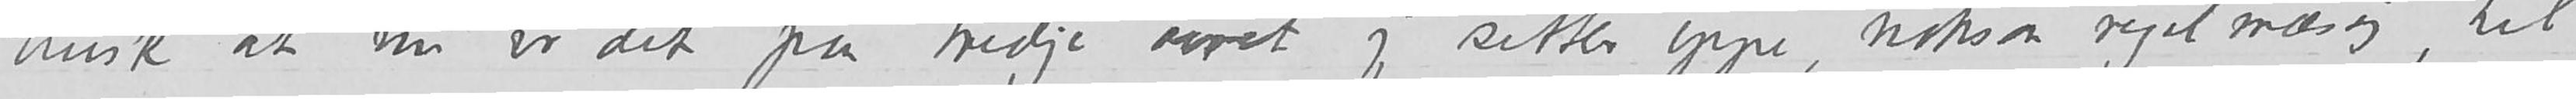husk at nu er det paa tredje aaret jeg sitter oppe, noksaa regelmæsig, til
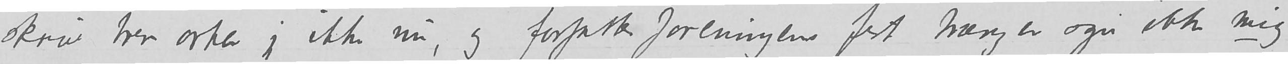skrive brev orker jeg ikke nu, og forfatterforeningens fest trænger ogsaa ikke mig.
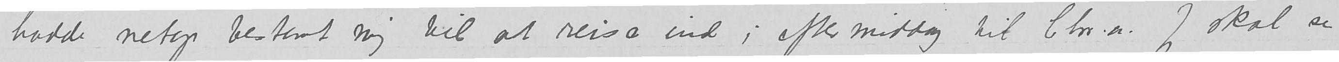hadde netop bestemt mig til at reise ind i eftermiddag til Chr.a. Jeg skal se
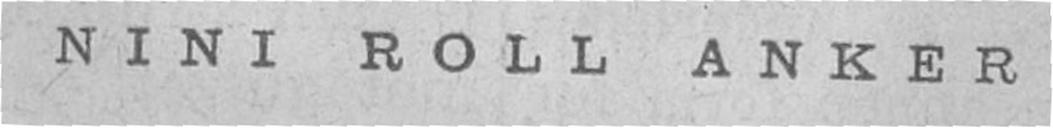NINI ROLL ANKER
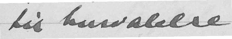til hurvælelse
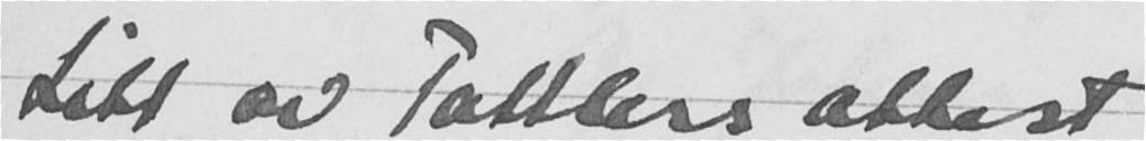Litt og Totthrs attest
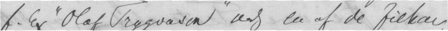f. ex. "Olaf Trygvason" en af de filhar-
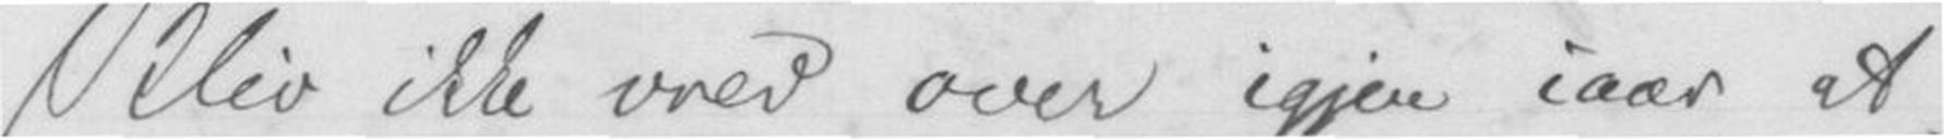Bliv ikke vred over igjen iaar at
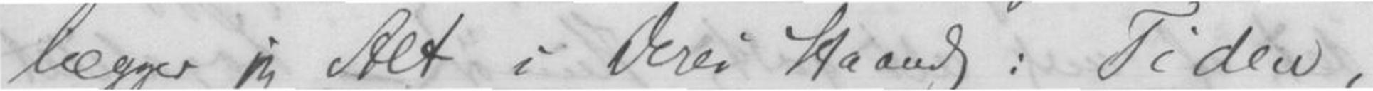lægger jeg Alt i Deres Haand: Tiden,
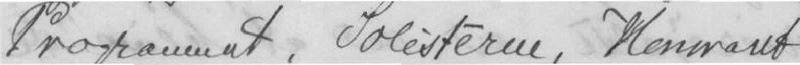Programmet, Solisterne, Honoraret
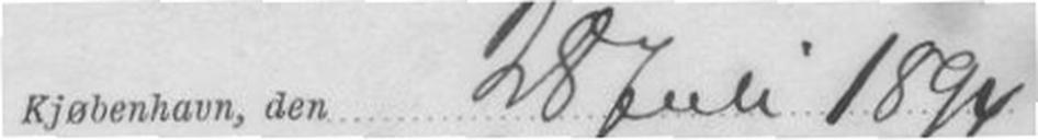Kjøbenhavn, den 28 Juli 1894
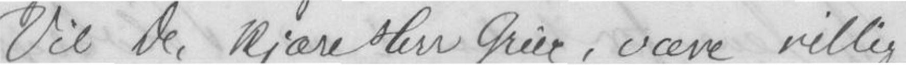Vil De, kjære Herr Grieg, være villig
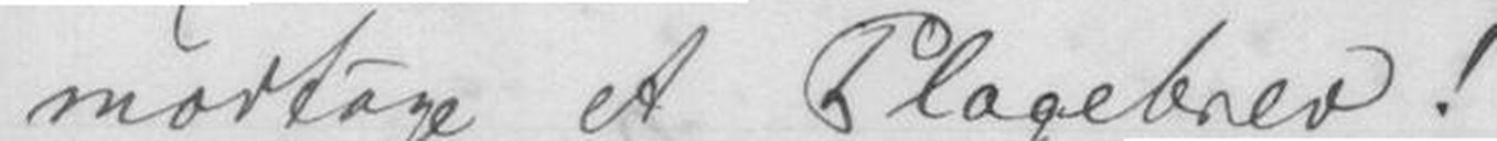modtage et Plagebrev !
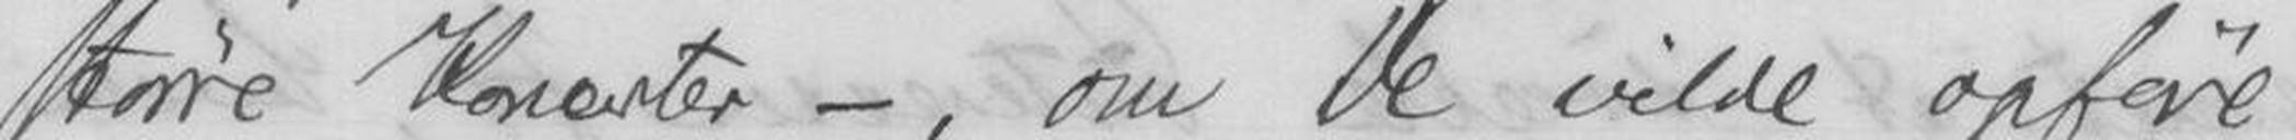større Koncerter-, om De vilde opføre
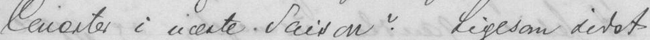Concerter i næste Saison ? Ligesom sidst
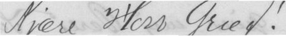Kjære Herr Grieg !
